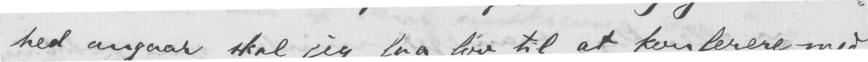hed angaar, skal jeg faa lov til at konferere med
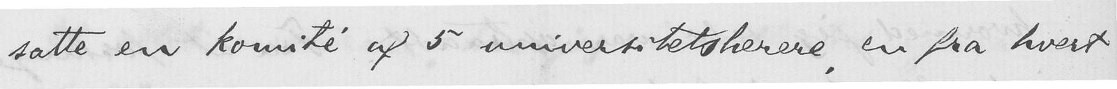satte en komité af 5 universitetslærere, en fra hvert
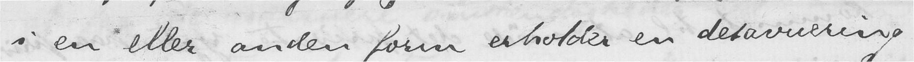i en eller anden form erholder en desavuering
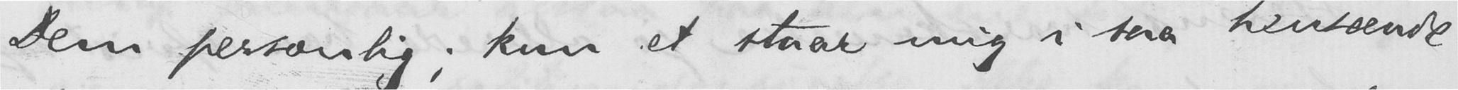Dem personlig; kun et staar mig i saa henseende
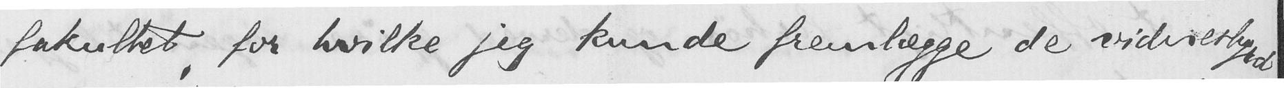fakultet, for hvilke jeg kunde fremlægge de vidnesbyrd,
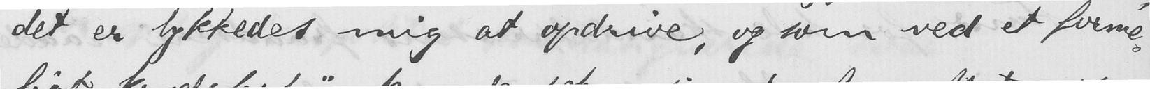det er lykkedes mig at opdrive, og som ved et forme-
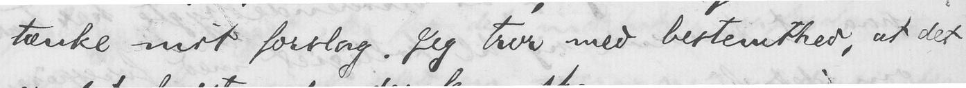tænke mit forslag. Jeg tror med bestemthed, at det
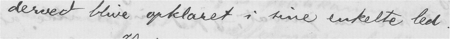derved blive opklaret i sine enkelte led.
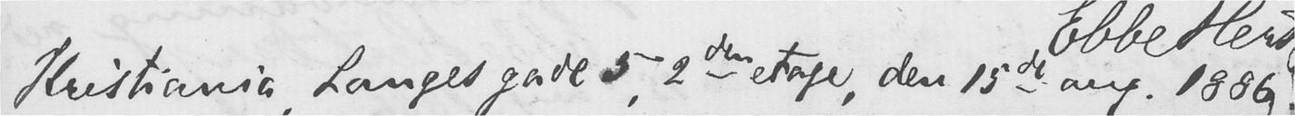Kristiania, Langes gade 5, 2den etage, den 15de aug. 1886.
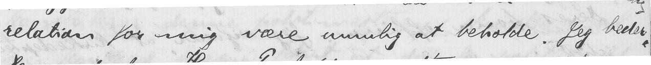relation for mig være umulig at beholde. Jeg beder
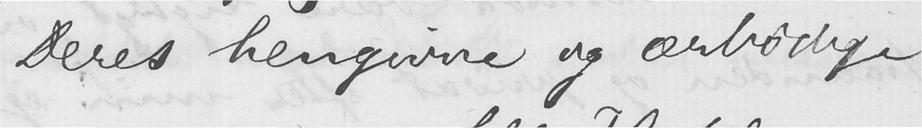Deres hengivne og ærbødige
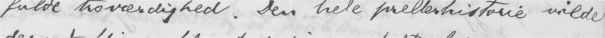fulde troværdighed. Den hele prellerhistorie vilde
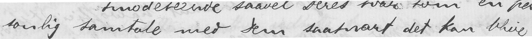sonlig samtale med Dem, saasnart det kan blive
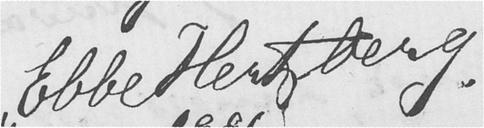Ebbe Hertzberg.
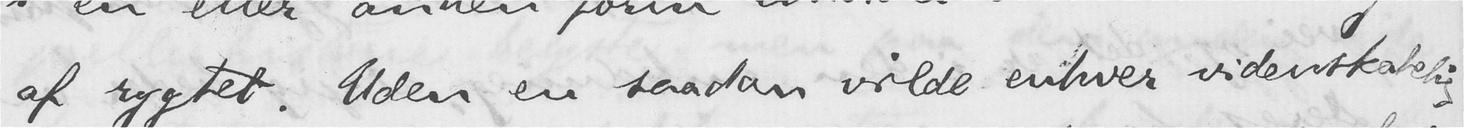af rygtet. Uden en saadan vilde enhver videnskabelig
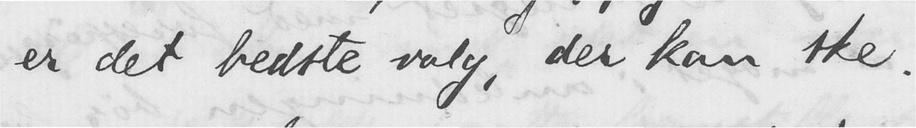er det bedste valg, der kan ske.
